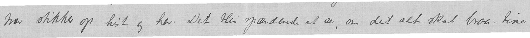trær stikker op hist og her. Det blir spændende at se, om det alt skal braa-tine
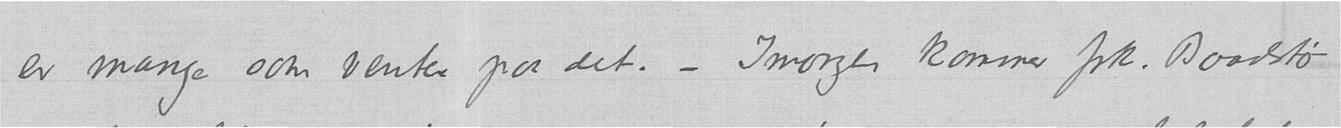er mange som venter paa det. – Imorgen kommer frk. Baadstø
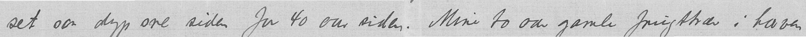set saa dyp sne siden for 40 aar siden. Mine to aar gamle frugttrær i haven
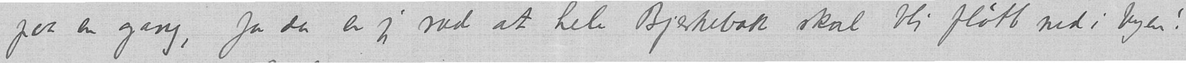paa en gang, for da er jeg ræd at hele Bjerkebæk skal bli fløtt ned i byen!
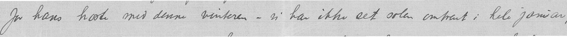for hans hoste med denne vinteren - vi har ikke set solen omtrent i hele januar,
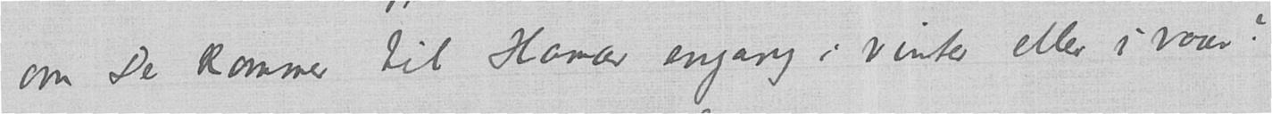om De kommer til Hamar engang i vinter eller i vaar?
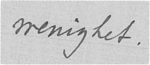menighet.
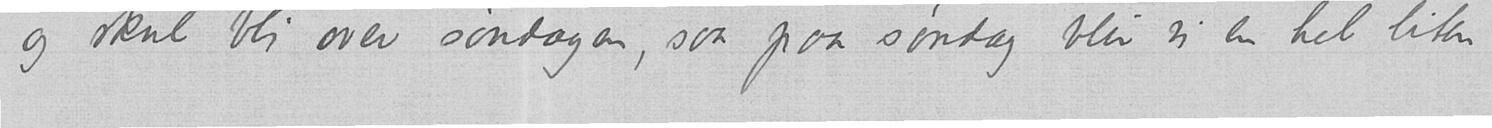og skal bli over søndagen, saa paa søndag blir vi en hel liten
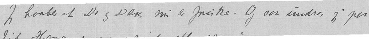Jeg haaber at De og Deres nu er friske. Og saa undres jeg paa
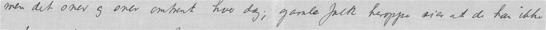men det sner og sner omtrent hver dag; gamle folk heroppe sier at de har ikke
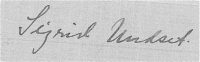Sigrid Undset.
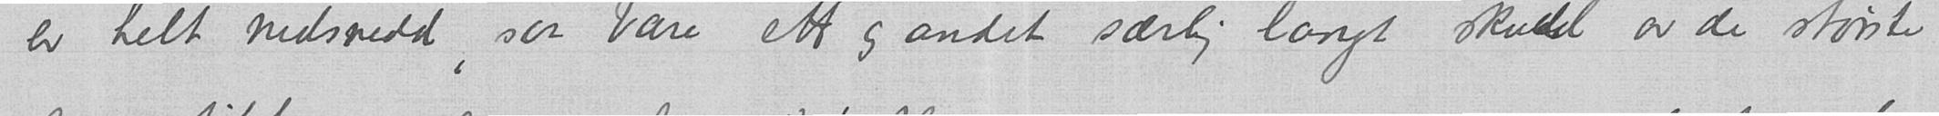er helt nedsnedd, saa bare ett og andet særlig langt skudd av de største
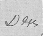Deres
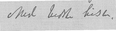Med bedste hilsen,
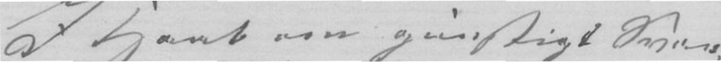I Haab om gunstigt Svar
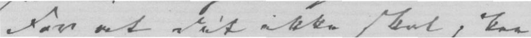For at det ikke skal skee
